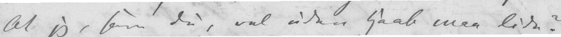At jeg, som du, vel uden Haab maa lide?
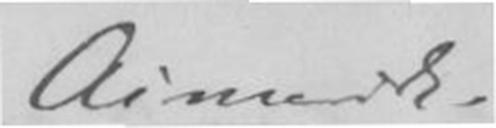Aimerik.
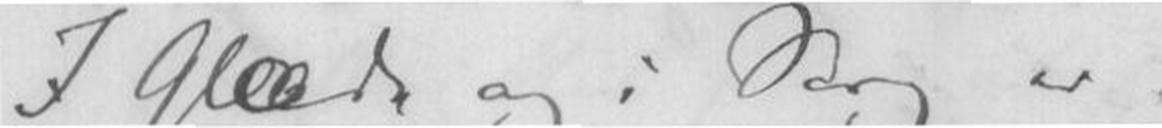I Glæde og i Sorg er
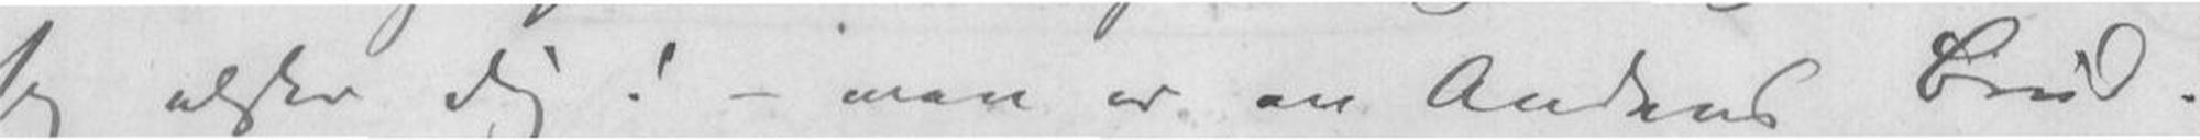Jeg ælsker dig! - men er en Andens Brud.
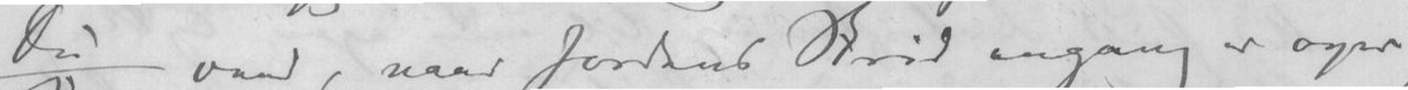Du vand, naar Jordens Strid engang er over,
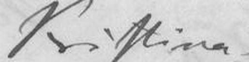Kristina.
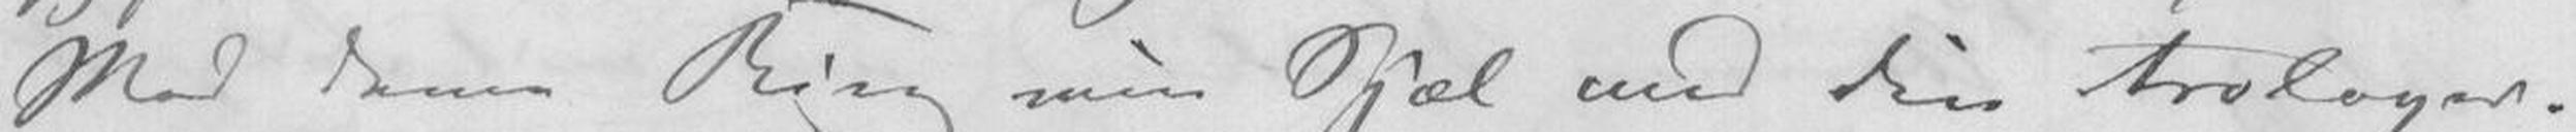Med denne Ring min Sjæl med din trolover.
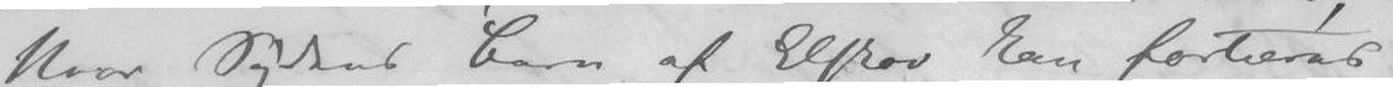Hvor Sydens Barn af Elskov kan fortæres,
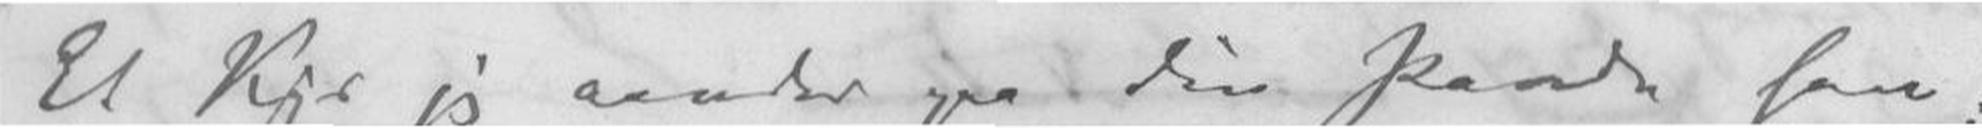Et Kys jeg aander paa din Pande hen;
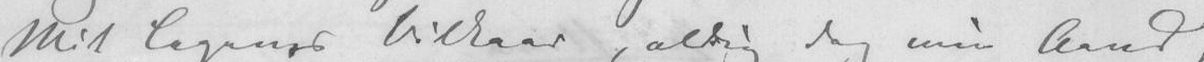Mit Legems Vilkaar, aldrig dog min Aand,
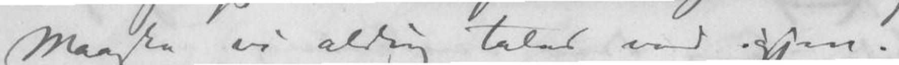Maaske vi aldrig tales ved igjen.
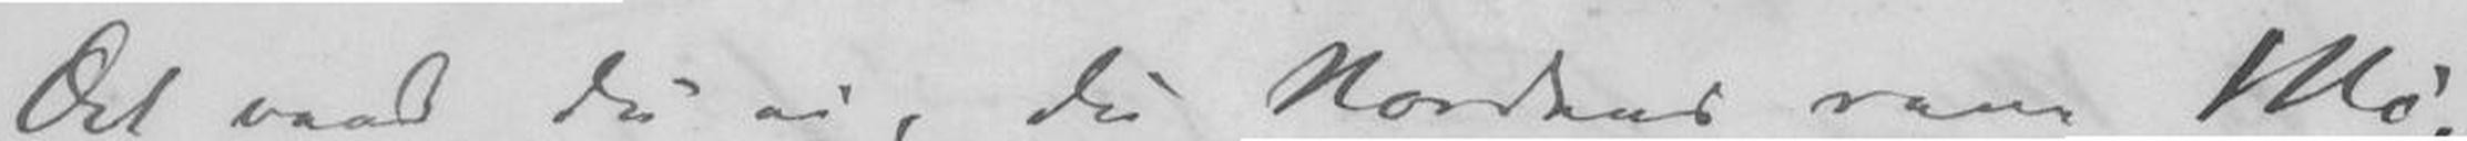Det veed du ei, du Nordens vene Mø,
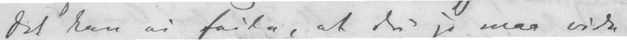Det kan vi feide, at du jo maa vide,
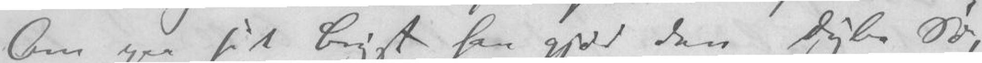Som paa sit Bryst han gjør den dybe Sø,
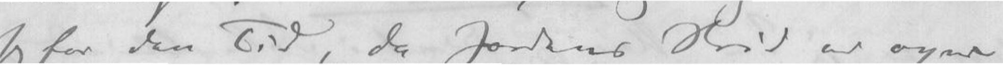Jeg for den Tid, da Jordens Strid er over
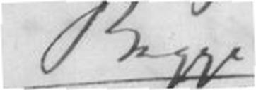Begge.
